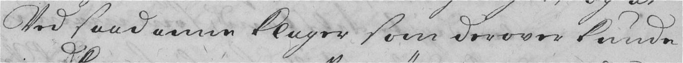Ved saadanne Klager som derover kunde
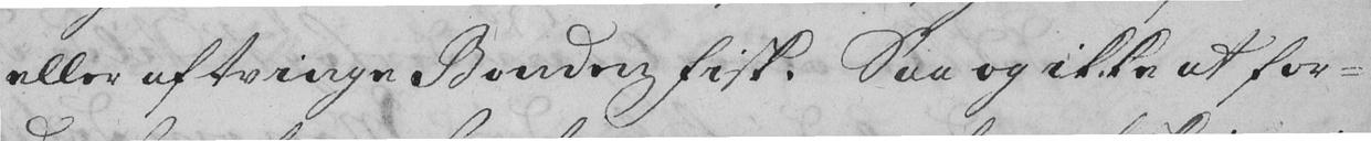eller aftvinge Bonden Fisk. Saa og ikke at for-
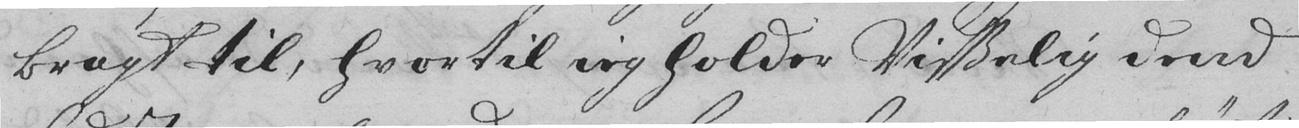bragt til, hvortil ieg holder Visselig dend
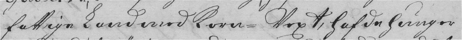fattige Land med Korn-Vext, hafde hunger
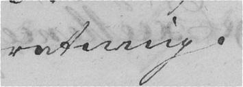retning.
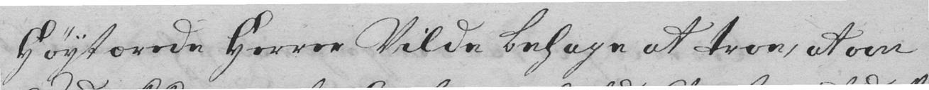Høytærede Herrer Vilde behage at troe, at om
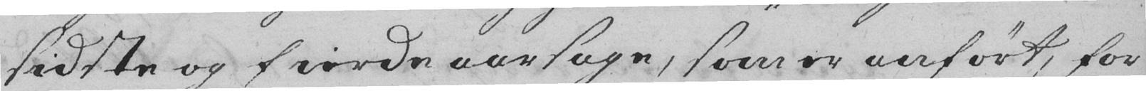sidste og fierde aarsage, som er anført, for
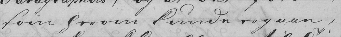som herom kunde ergaae,
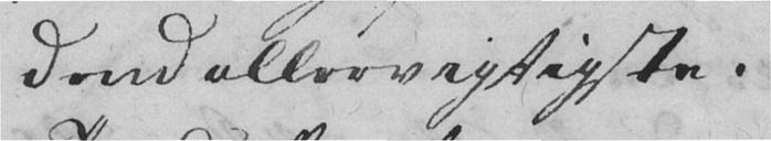dend allervigtigste.
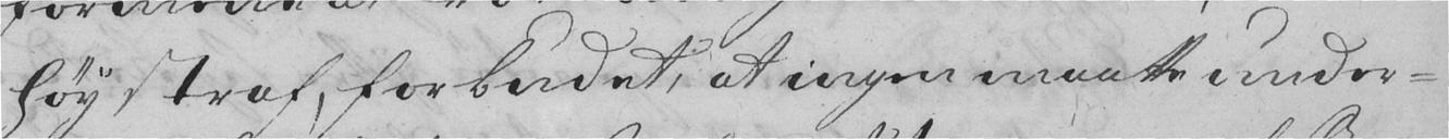høy straf, forbudet, at ingen maatte under-
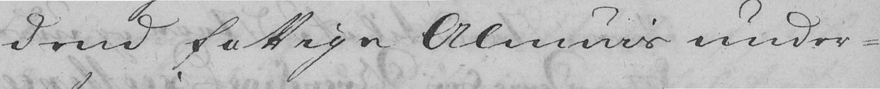dend fattige Almuis under-
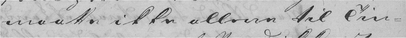maatte ikke allene til Tin-
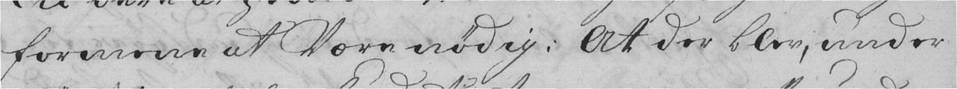formene at Være nødig: At der blev, under
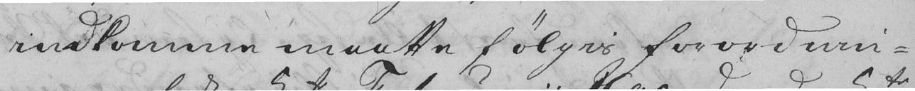indkomme maatte følgis forordnin-
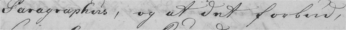Paragraphus, og at det forbud,
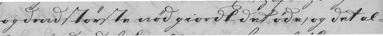og dend største nød giordt det øde, og det al-
